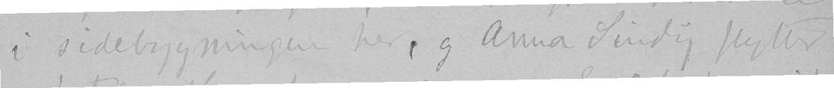i sidebygningen her, og Anna Sindig flytter
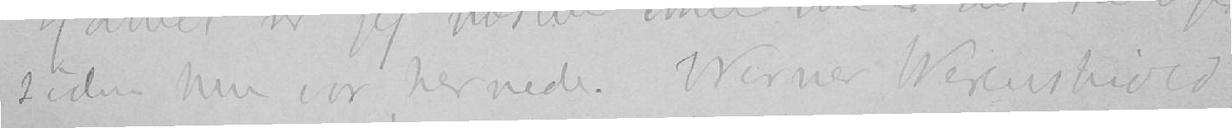siden hun var hernede. Werner Werenskiold
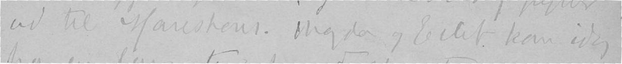ud til Mareskous. Magda og Eilif kom idag
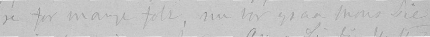se for mange folk, nu bor ogsaa Mons Lie
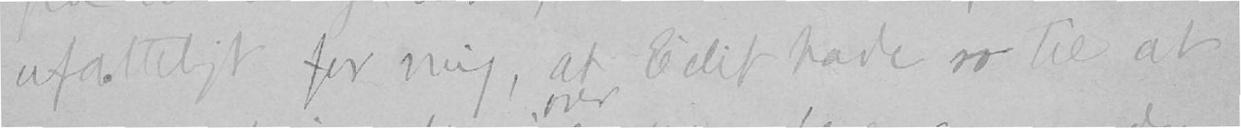ufatteligt for mig, at Eilif havde ro til at
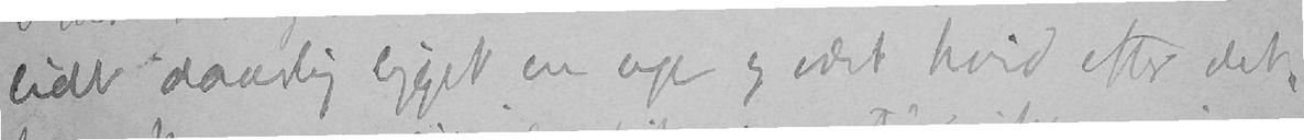lidt daarlig ligget en uge og været hvid efter det,
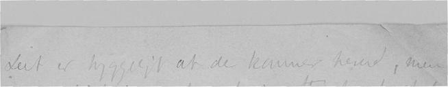Det er hyggeligt at de kommer herud, men
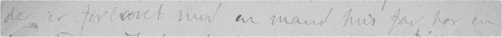der er forlovet med en mand hvis far har en
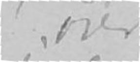over
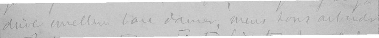drive imellem bare damer, mens hans arbeide
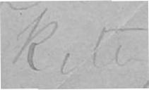Kitty.
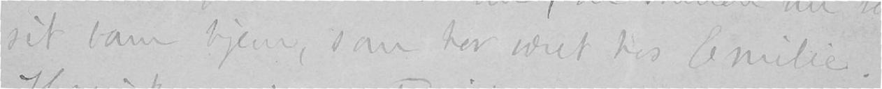sit barn hjem, som har været hos Emilie.
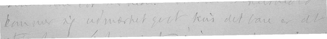kommer sig udmærket godt, hvis det bare er at
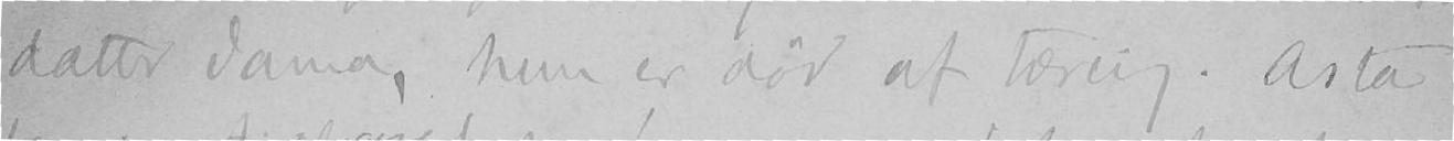datter Janna, hun er død af tæring. Asta
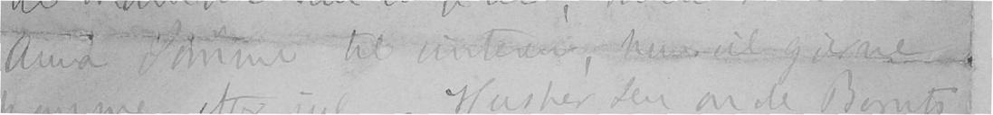Anna Sømme til vinteren, hun vil gjerne
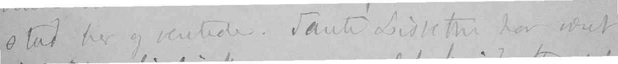stod her og ventede. Tante Lisbeth har været
